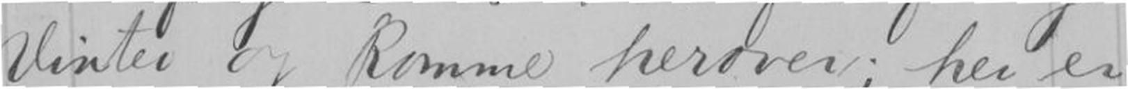Vinter og komme herover; her er
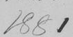1881
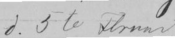d. 5te Februar
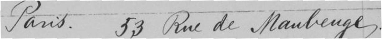Paris.53 Rue de Maubenge.
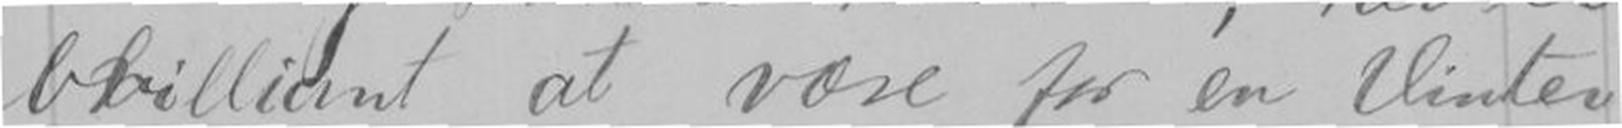brilliant at være for en Vinter,
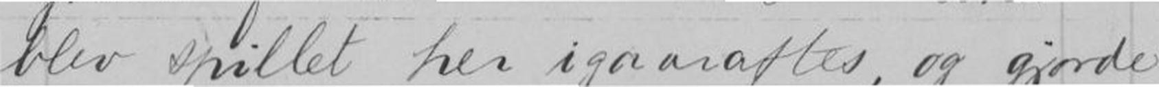blev spillet her igaaraftes, og gjorde
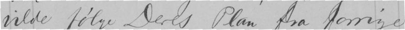vilde følge Deres Plan fra forrige
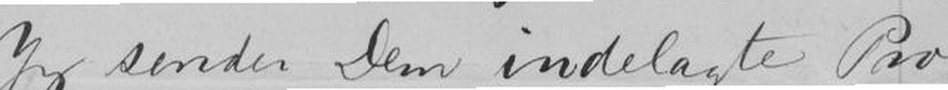Jeg sender Dem indelagte Pro-
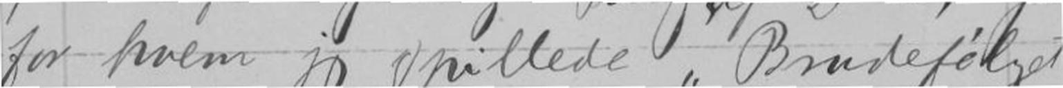for hvem jeg spillede Brudefølget
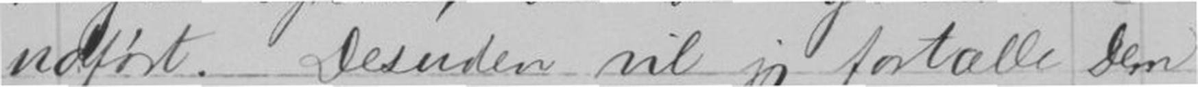udført. Desuden vil jeg fortælle Dem
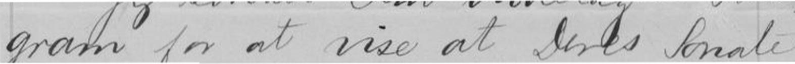gram for at vise at Deres Sonate
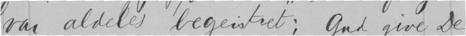var aldeles begeistret; Gud give De
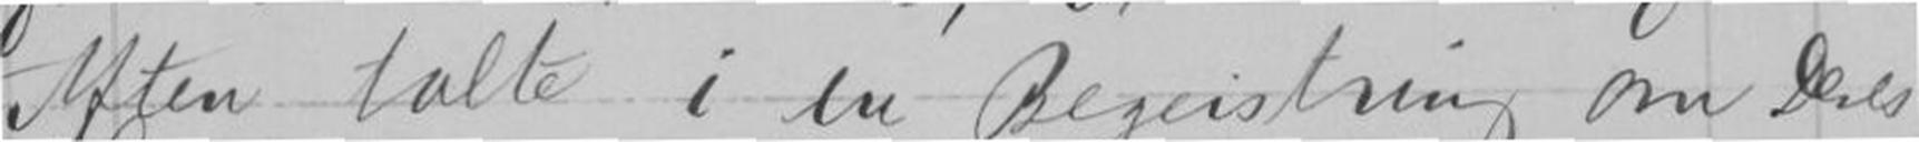Aften talte i en Begeistring om Deres
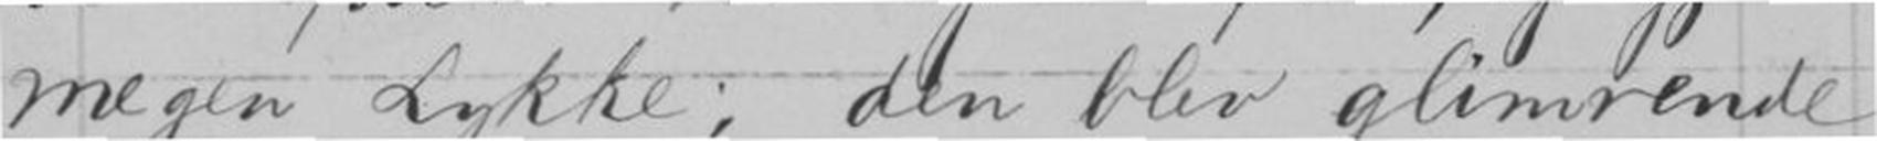megen Lykke; den blev glimrende
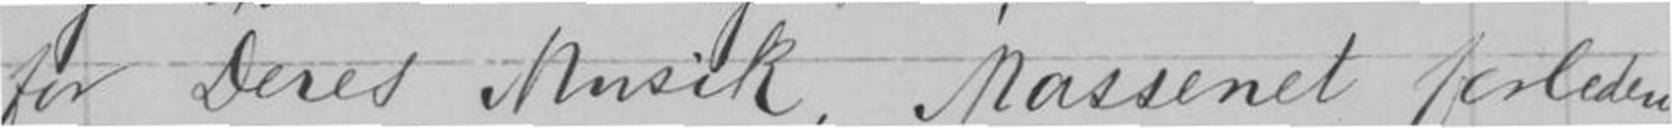for Deres musik, Massenet forleden
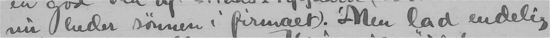nu heder sønnen i firmaet). Men lad endelig
